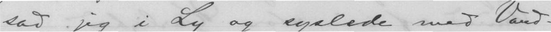sad jeg i Ly og syslede med Vand-
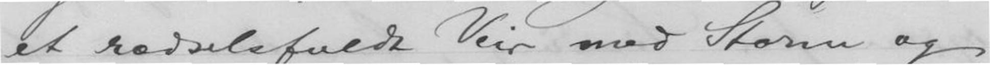et rædselfuldt Veir med Storm og
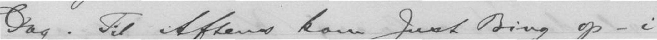Dag. Til Aftens kom Just Bing op - i
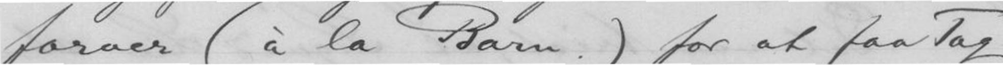farver (à la Barn) for at faa Tag
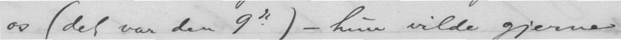os (det var den 9de) - hun vilde gjerne
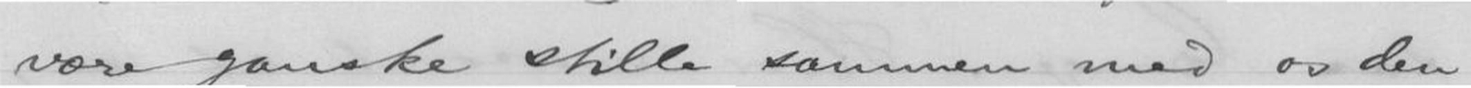være ganske stille sammen med os den
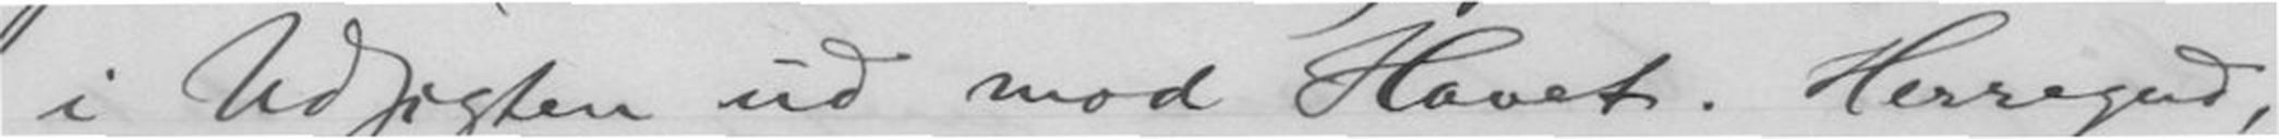i Udsigten ud mod Havet. Herregud,
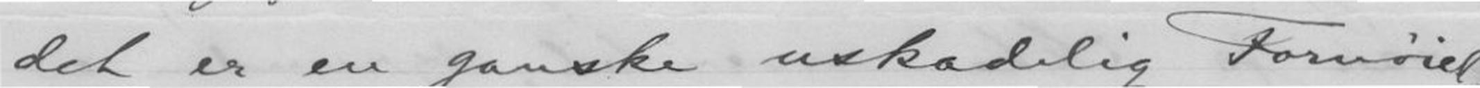det er en ganske uskadelig Fornøiel-
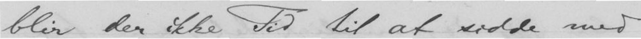blir der ikke Tid til at sidde med
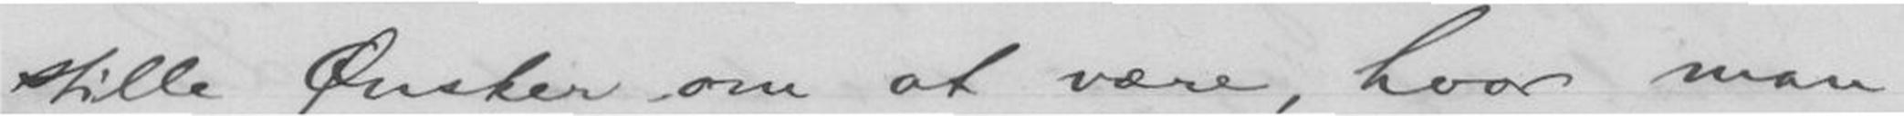stille Ønsker om at være, hvor man
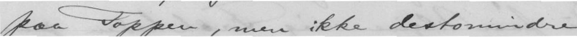paa Toppen, men ikke destomindre
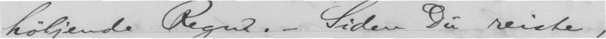høljende Regn. - Siden Du reiste,
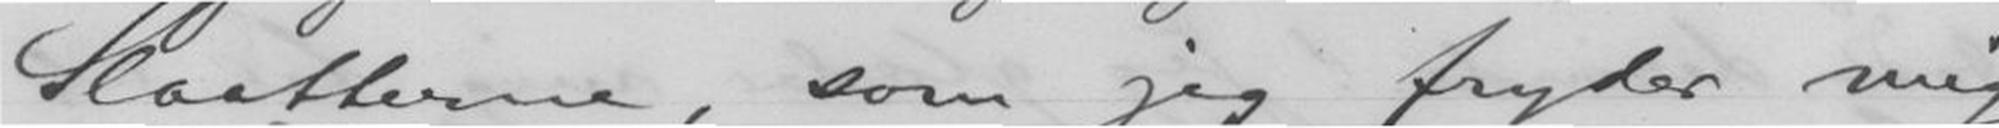Slaaterne, som jeg fryder mig
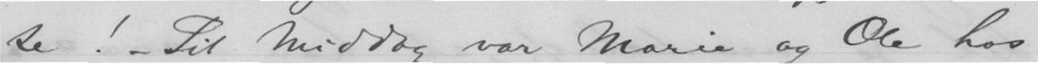se! - Til Middag var Marie og Ole hos
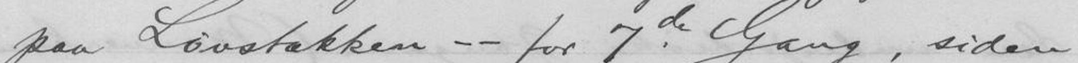paa Løvstakken -- for 7de Gang, siden
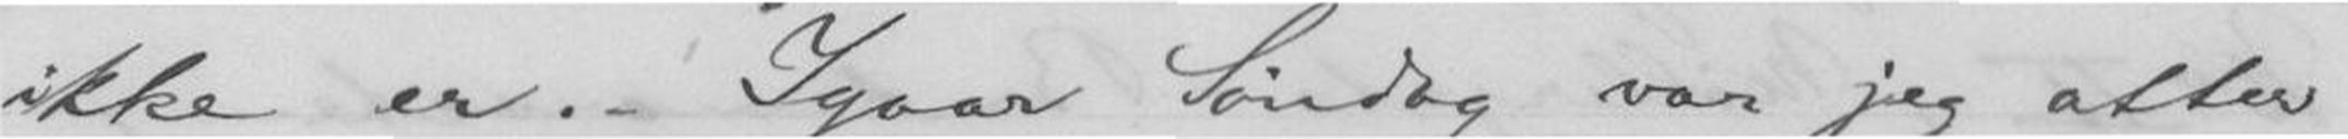ikke er. - Igaar Søndag var jeg atter
